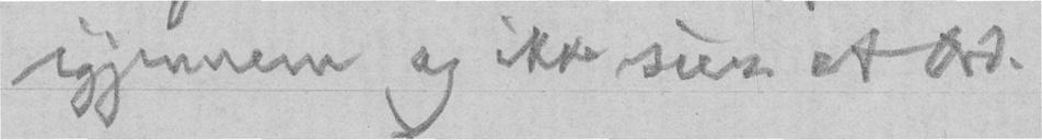igjennem og ikke sier et Ord.
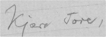Kjære Tore,
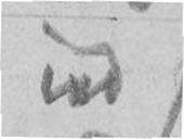ud
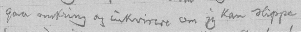gaa omkring og inkvirere om jeg kan slippe
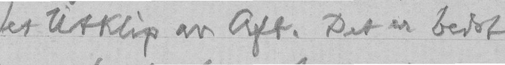et Utklip av Aft. Det er bedst
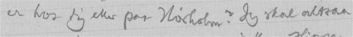er hos dig eller paa Nørholm? Jeg skal altsaa
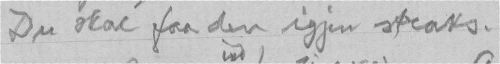Du skal faa den igjen straks.
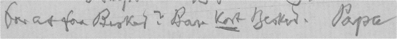for at faa Besked? Bare kort Besked. Papa
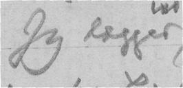Jeg lægger,
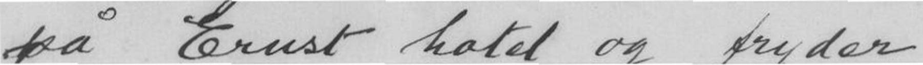på Ernst hotel og fryder
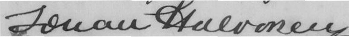Johan Halvorsen
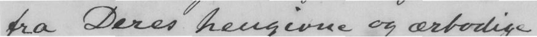fra Deres hengivne og ærbødige
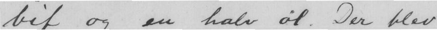bif og en halv øl. Der blev
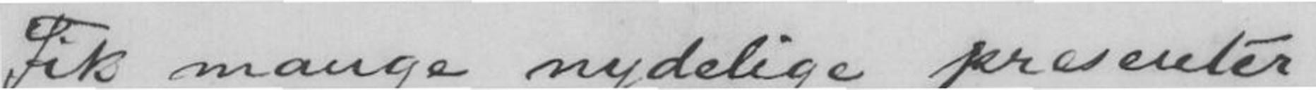Fik mange nydelige presenter
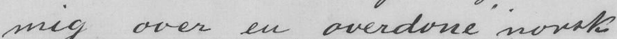mig over en overdone norsk
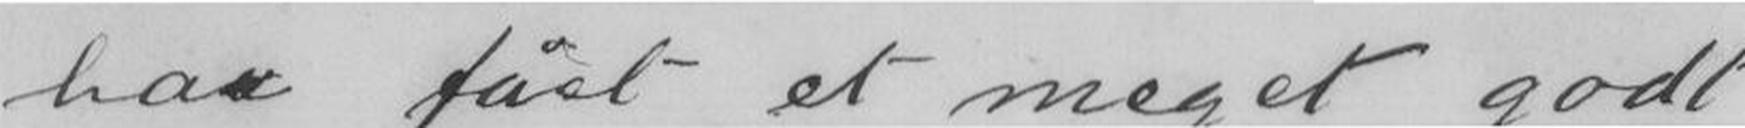har fået et meget godt
